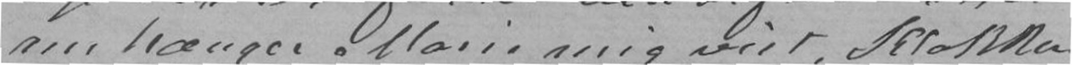nu hænger Marie mig vist. Klokken
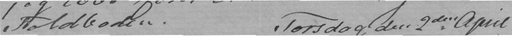Toldboden. Torsdag den 2den April
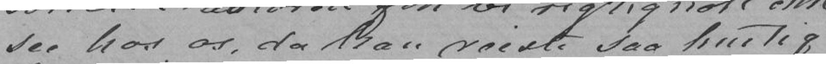see hos os, da han reiste saa hurtig
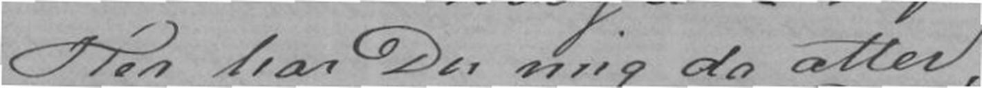Her har Du mig da atter,
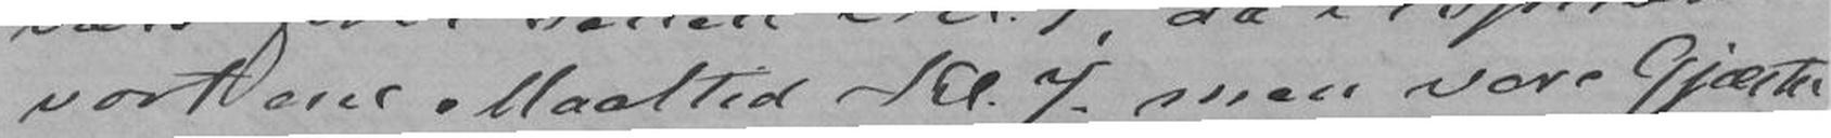vort ene Maaltid Kl.7, men vore Gjæster
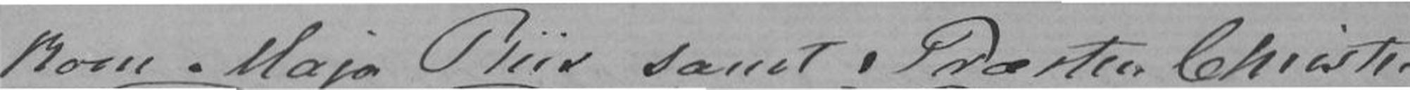kom Major Riis samt Præsten Christie
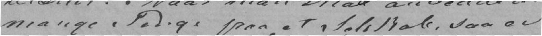mange Penge paa et Selskab, saa er
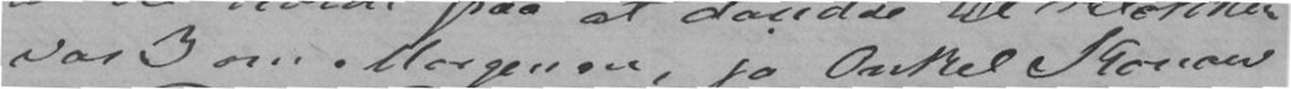var 3 om Morgenen, jo Onkel Konow
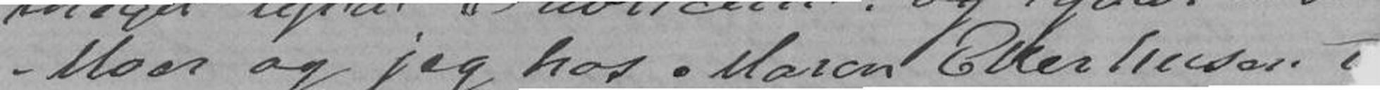Moer og jeg hos Maren Ellerhusen
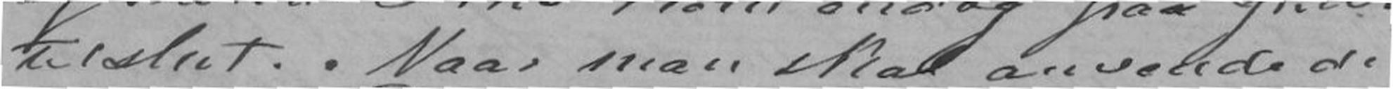tilslut. Naar man skal anvende de
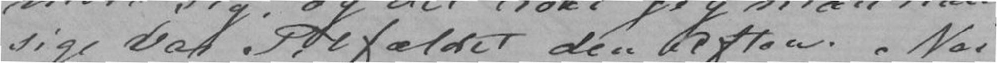sige var Tilfældet den Aften. Nei
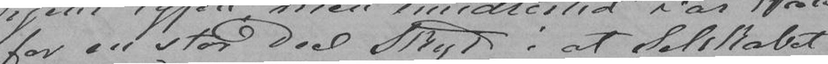for en stor Deel Skyld i at Selskabet
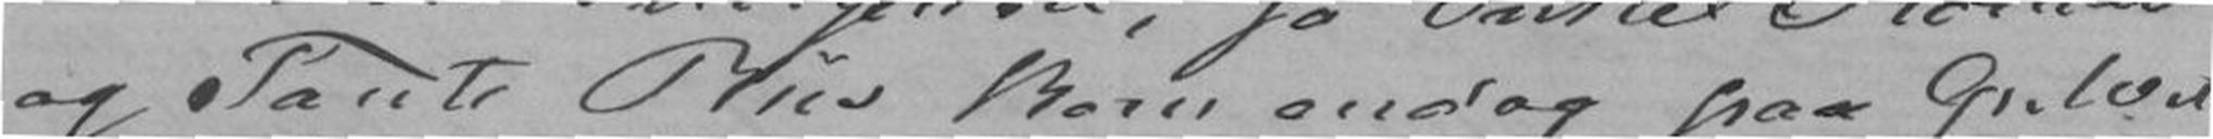og Tante Riis kom endog paa Gulvet
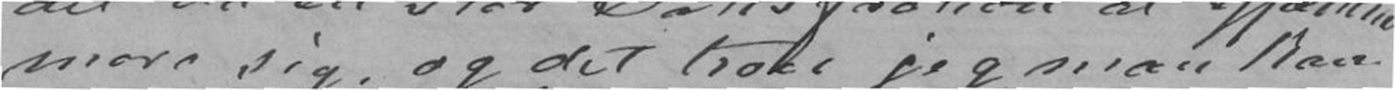more sig, og det troer jeg man kan
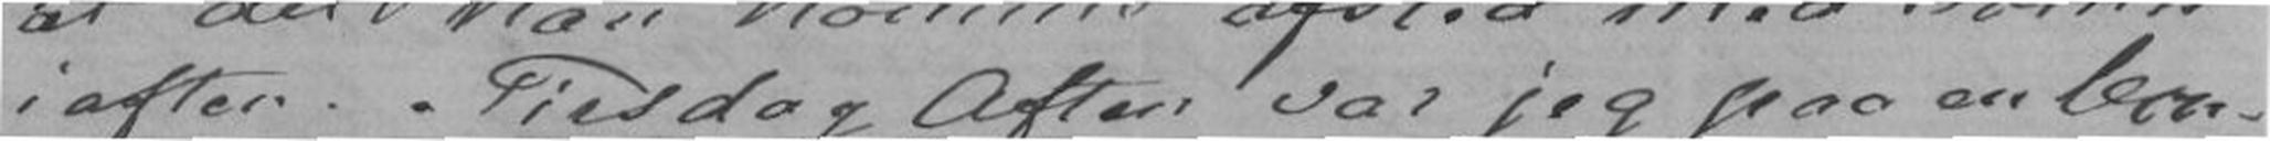i aften. Tisdag Aften var jeg paa en Con-
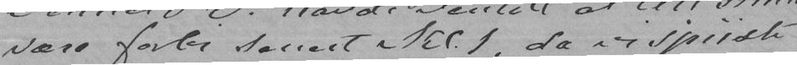være forbi senest Kl 1, da vi spiiste
